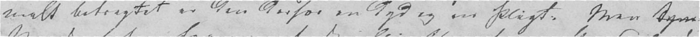melt betragtet er den derfor en Dyd og en Pligt. Men syne
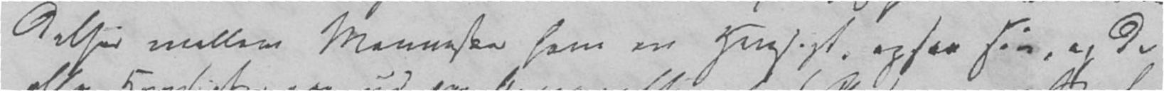delser mellem Mennesker som en Hensigt, ogsaa sin, og da
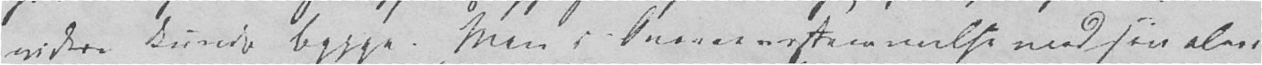videre kunde bygge. Men i Overeensstemmelse med sin alm.
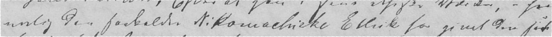melig den saakaldte Nikomachiske Ethik har givet den sit
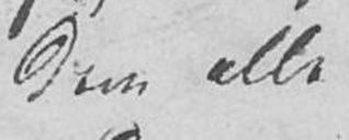dem alle
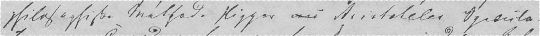philosophiske Methode klipper om Aristoteles Specula-
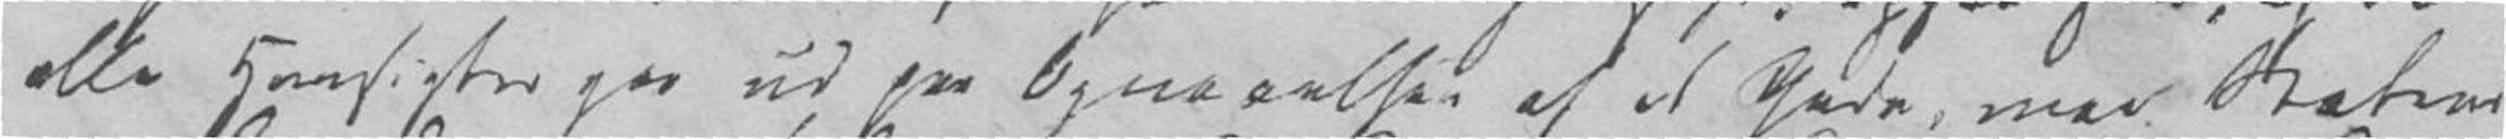alle Hensigter gaa ud paa Opnaaelsen af et Gode, maa Statens
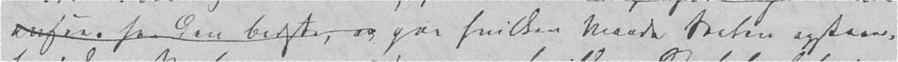ansees for den bedste, og paa hvilken Maade Staten opstaaer,
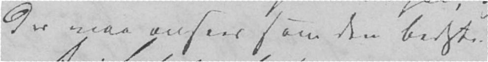der maa ansees som den bedste.
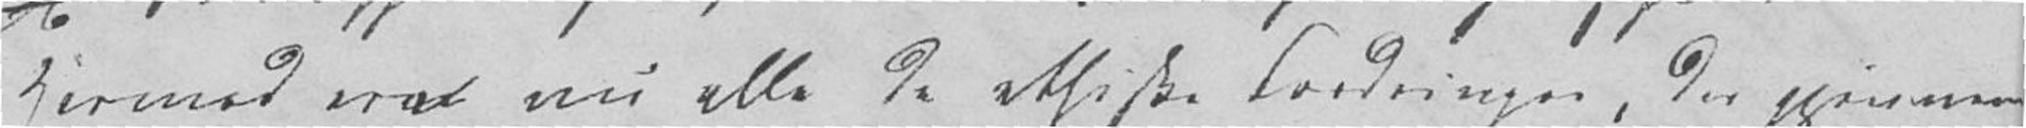Hermed var nu alle de ethiske Fordringer, der gjennem
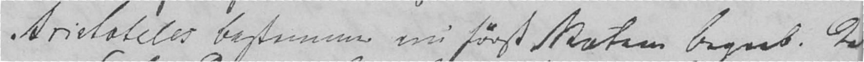Aristoteles bestemmer nu først Statens Begreb. Den
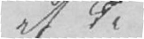af de
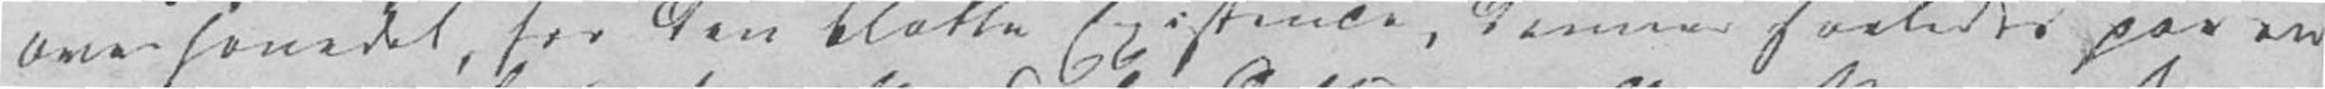overhovedet, for den blotte Existence, danner saaledes paa en
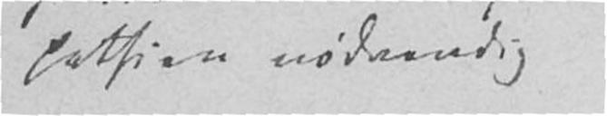pathien nødvendig
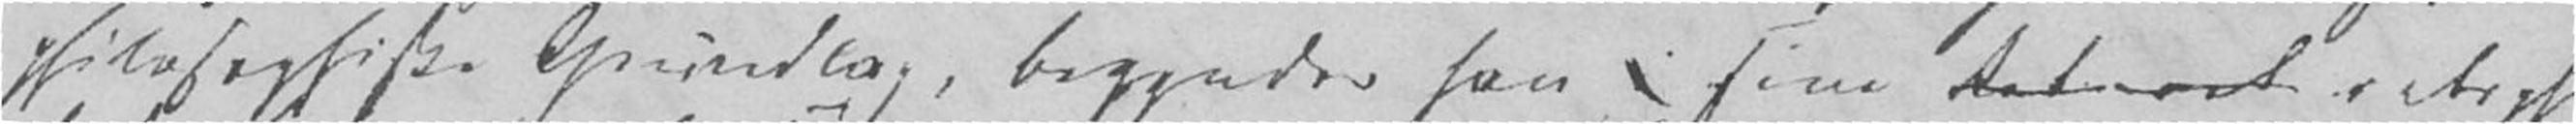philosophiske Grundlag, begynder han sine retsphi-
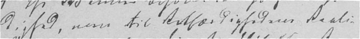dighed, men til Retfærdighedens Reali-
